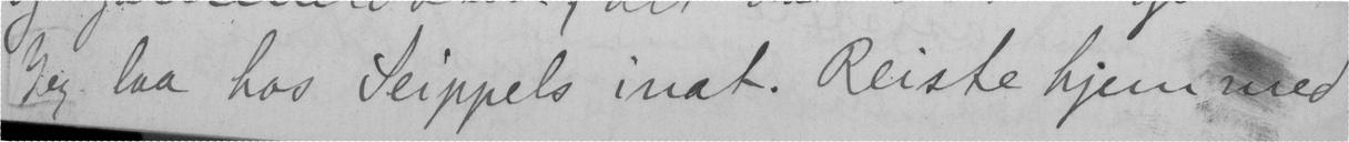Jeg laa hos Seippels inat. Reiste hjem med
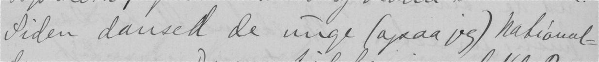Siden dansed de unge (ogsaa jeg) national-
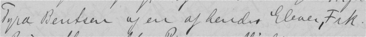Tyra Bentsen og en af hendes Elever, Frk.
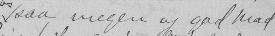saa megen og god mad
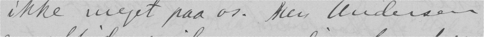ikke meget paa os. Men Andersen
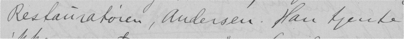Restauratøren, Andersen. Han tjente
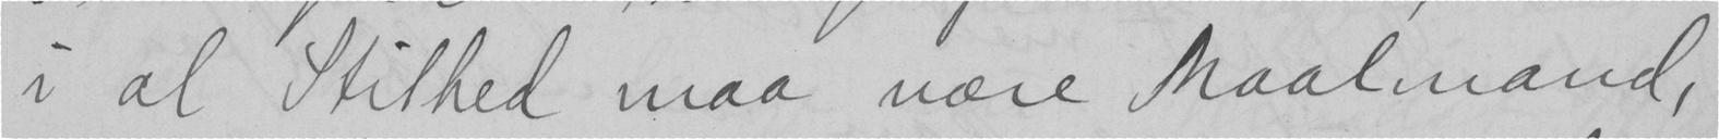i al Stilhed maa være maalmand,
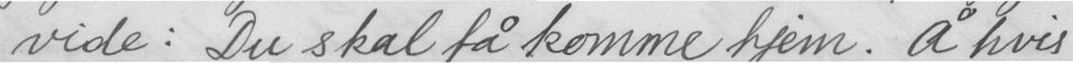vide: Du skal få komme hjem. Å hvis
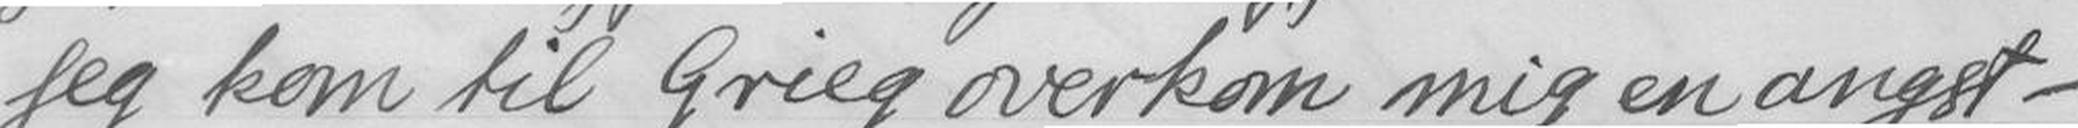jeg kom til Grieg overkom mig en angst -
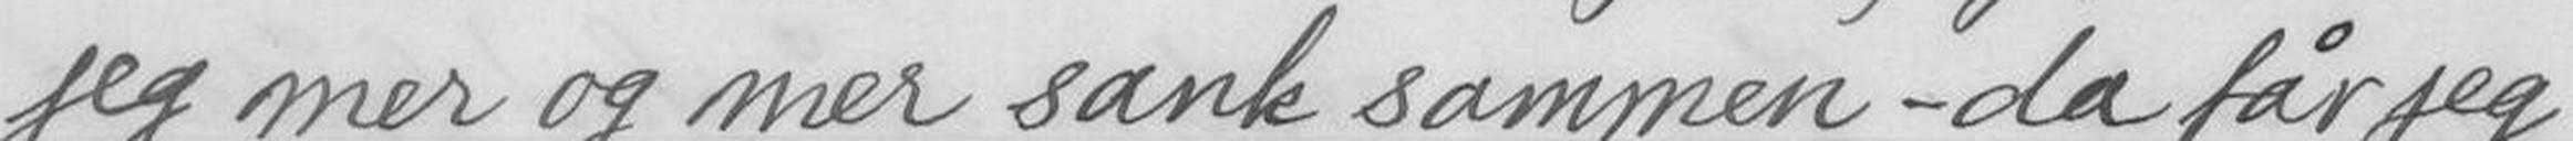jeg mer og mer sank sammen - da får jeg
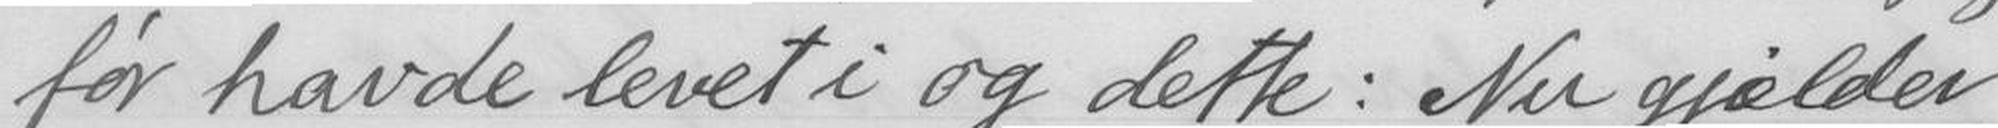før havde levet i og dette: Nu gjælder
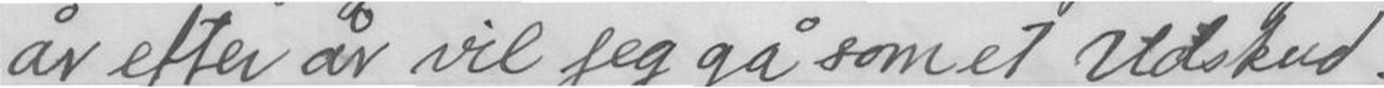år efter år vil jeg gå som et Udskud.
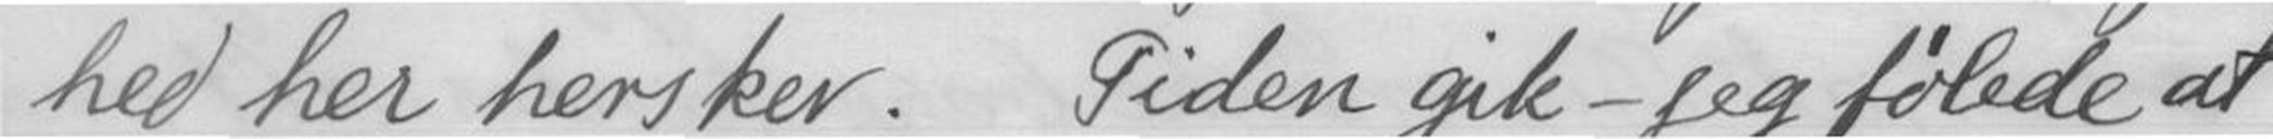hed her hersker. Tiden gik - jeg følede at
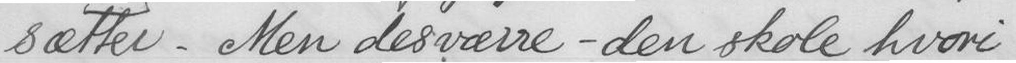sætter. Men desværre - den skole hvori
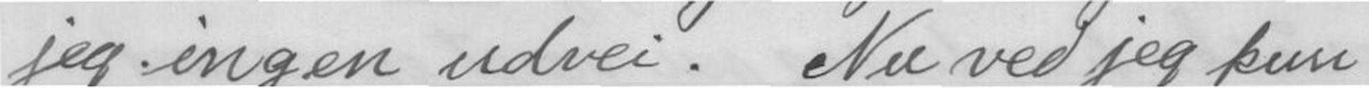jeg ingen udvei. Nu ved jeg kun
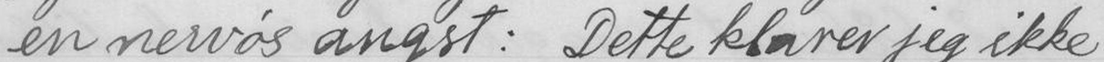en nervøs angst: Dette klarer jeg ikke
